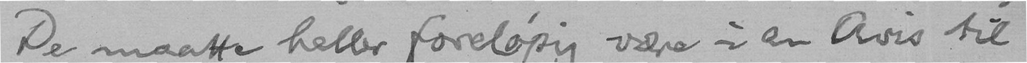De maatte heller foreløpig være i en Avis til
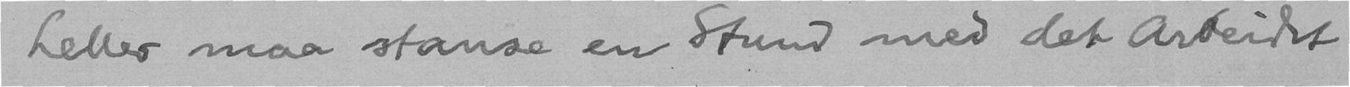heller maa stanse en Stund med det Arbeidet
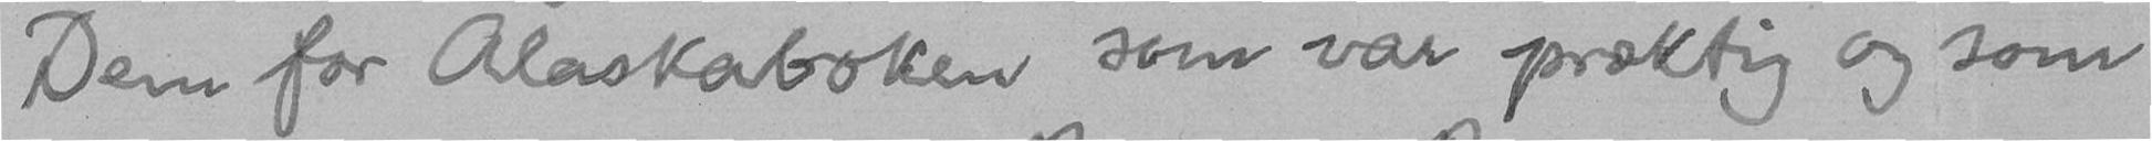Dem for Alaskaboken som var præktig og som
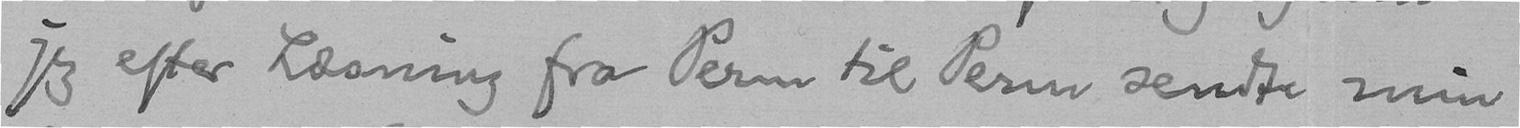jeg efter Læsning fra Perm til Perm sendte min
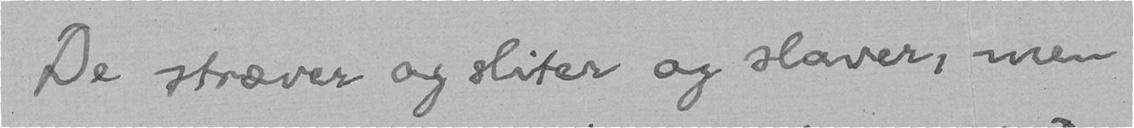De stræver og sliter og slaver, men
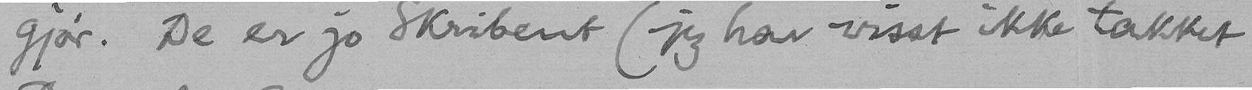gjør. De er jo Skribent (jeg har visst ikke takket
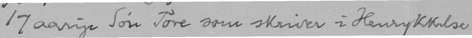17 aarige Søn Tore som skriver i Henrykkelse
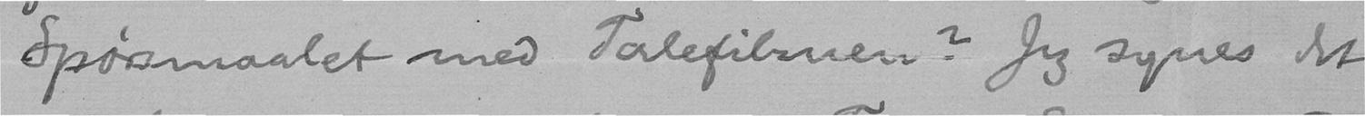Spørsmaalet med Talefilmen? Jeg synes det
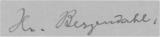Hr. Bergendahl,
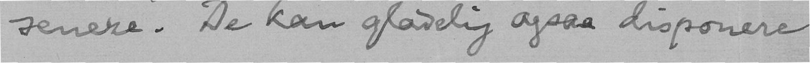senere. De kan gladelig ogsaa disponere
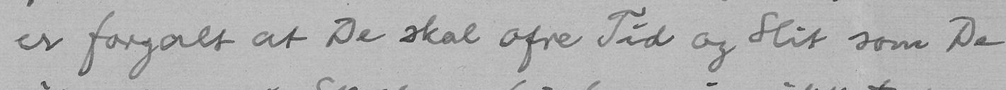er forgalt at De skal ofre Tid og Slit som De
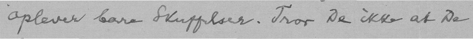oplever bare Skuffelser. Tror De ikke at De
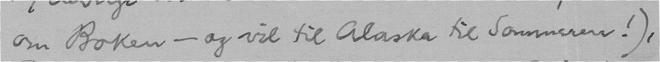om Boken - og vil til Alaska til Sommeren!),
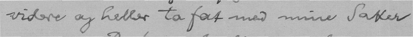videre og heller ta fat med mine Saker
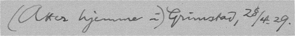(Atter hjemme i) Grimstad, 28/4. 29.
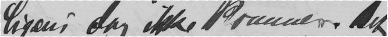ligens dog ikke kommer. Det
